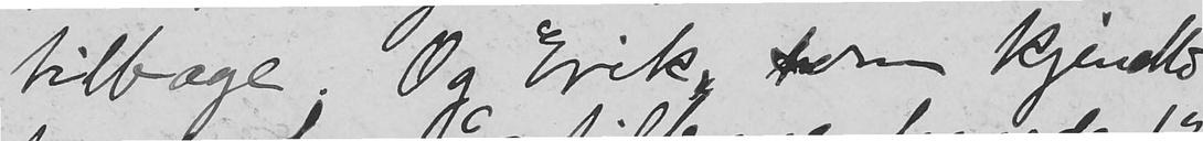tilbage. Og Erik som kjendte
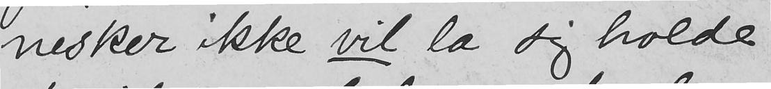nesker ikke vil la sig holde
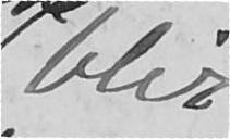blir
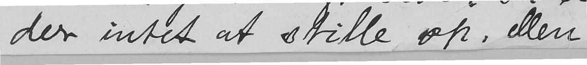der intet at stille op. Men
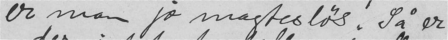er man jo magtesløs! Så er
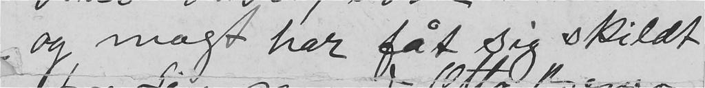og magt har fåt sig skildt
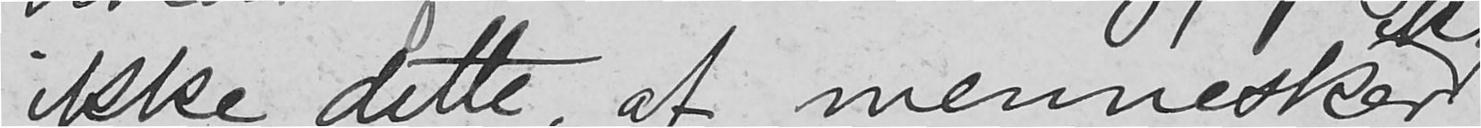ikke dette, at mennesker
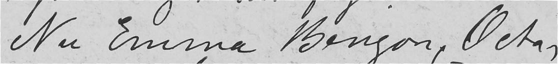Nu Emma Benzon, Octa-
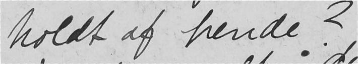holdt af hende?
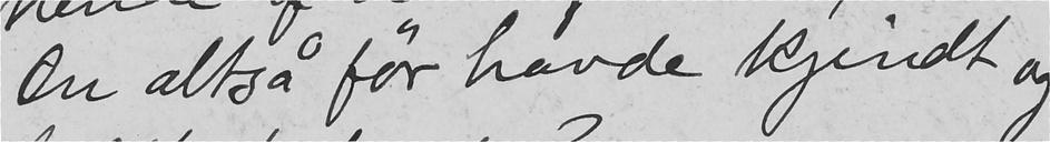Du altså før havde kjendt og
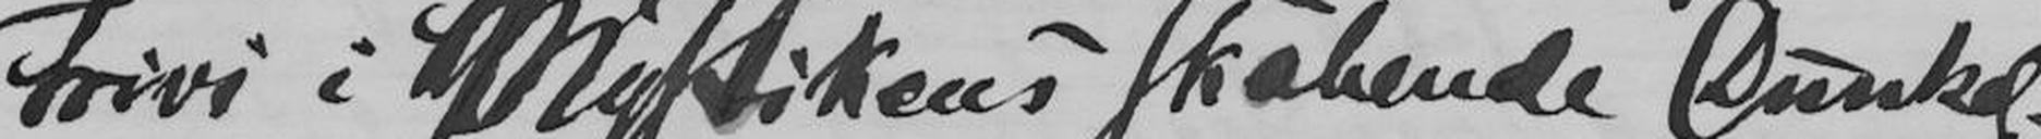trivs i Mystikens skabende Dunkel-
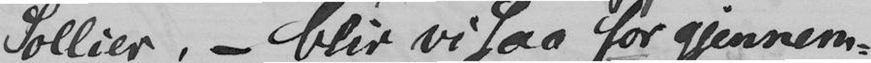Sollier, - blir vi saaa for gjennem-
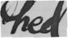hed.
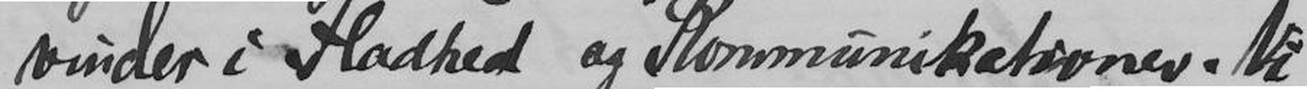vinder i Fladhed og Kommunikationer. Vi
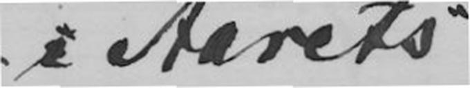i Aarets
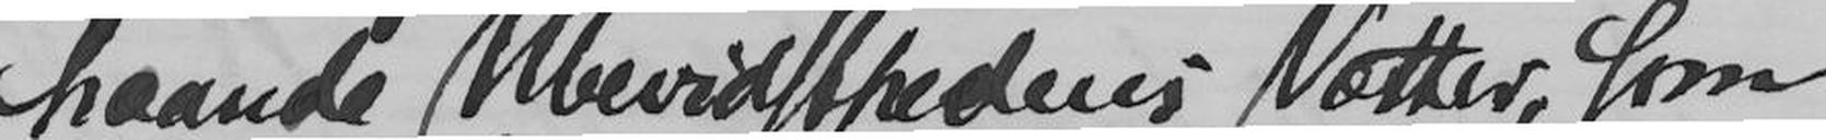haande Ubevidsthedens Nætter, som
